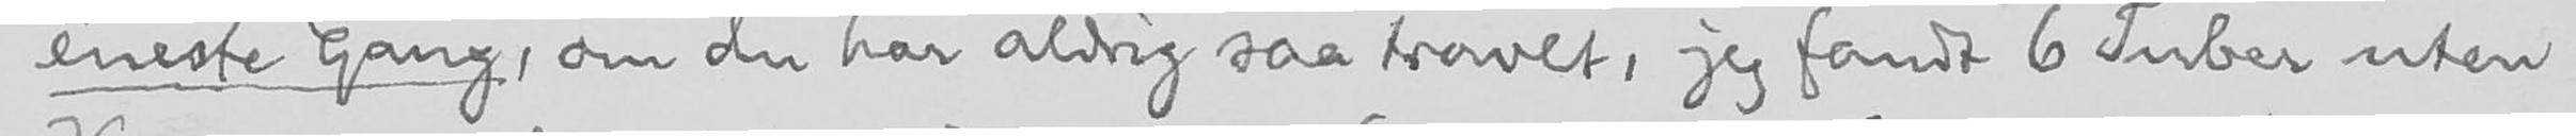eneste Gang, om du har aldrig saa travlt, jeg fandt 6 Tuber uten
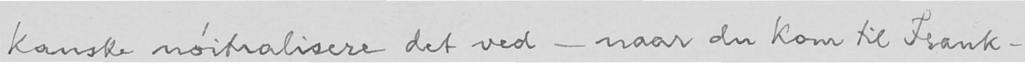kanske nøitralisere det ved - naar du kom til Frank-
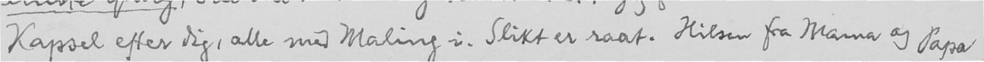Kapsel efter dig, alle med Maling i. Slikt er raat. Hilsen fra Mama og Papa
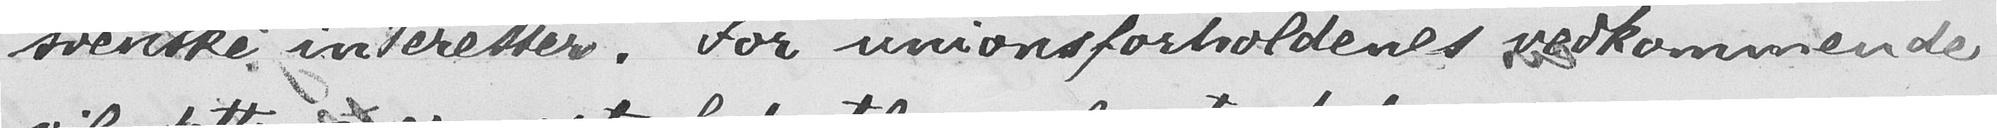svenske interesser. For unionsforholdenes vedkommende
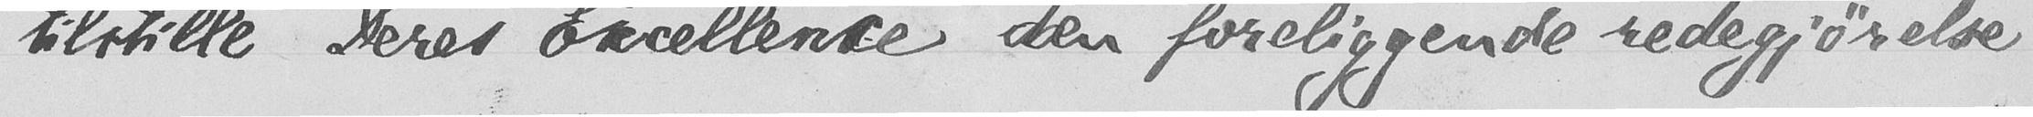tilstille Deres Excellense den foreliggende redegjørelse
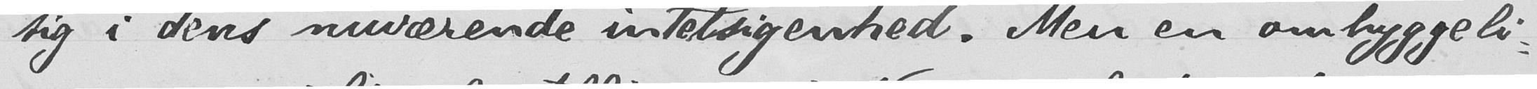sig i dens nuværende intetsigenhed. Men en omhyggeli-
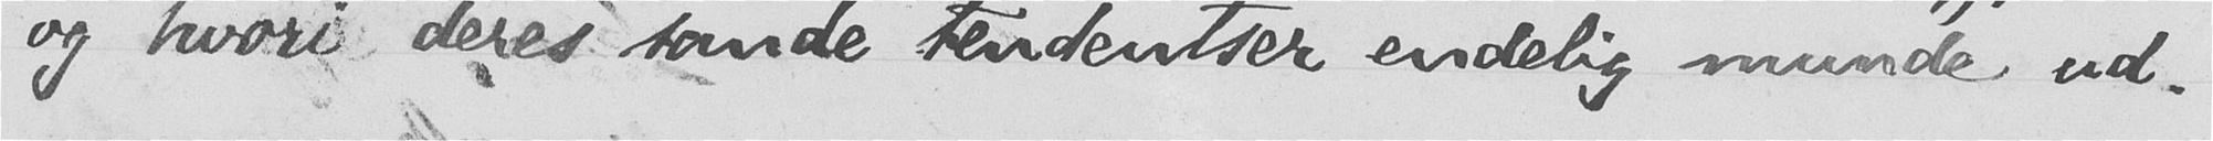og hvori deres sande tendentser endelig munde ud.
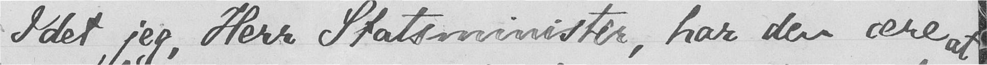Idet jeg, Herr Statsminister, har den ære at
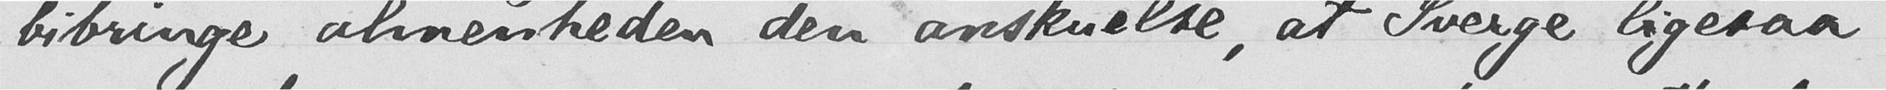bibringe almenheden den anskuelse, at Sverge ligesaa
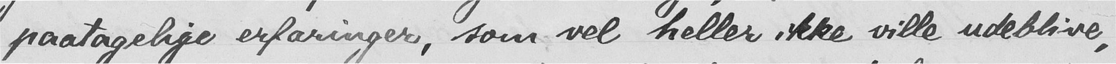paatagelige erfaringer, som vel heller ikke ville udeblive,
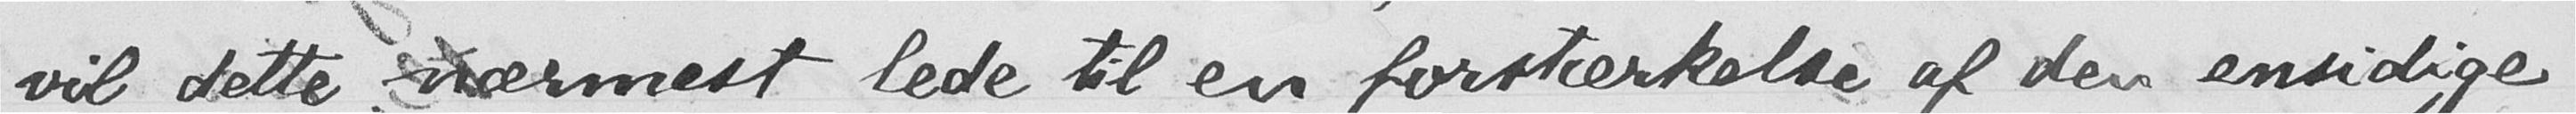vil dette nærmest lede til en forstærkelse af den ensidige
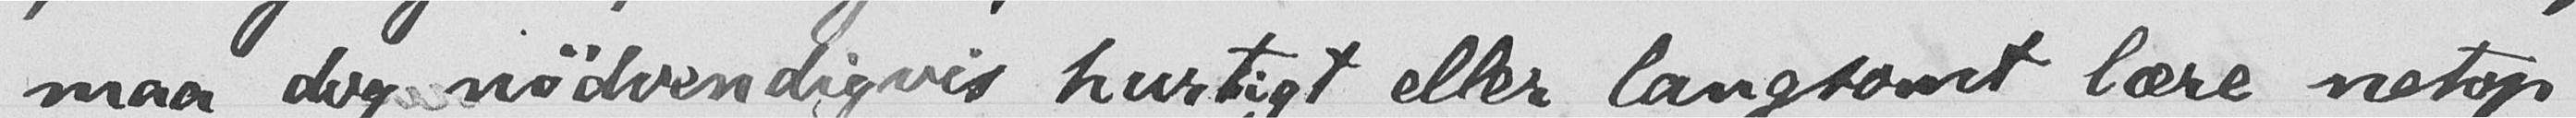maa dog nødvendigvis hurtigt eller langsomt lære netop
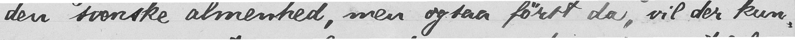den svenske almenhed, men ogsaa først da, vil der kun-
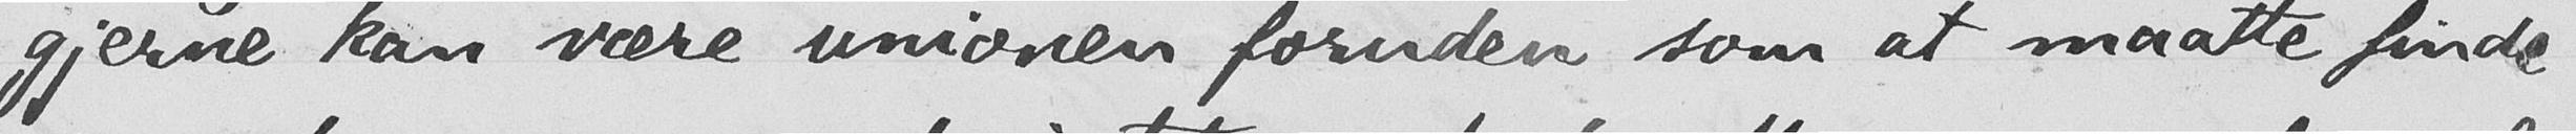gjerne kan være unionen foruden som at maatte finde
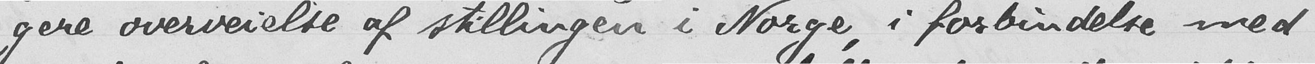gere overveielse af stillingen i Norge, i forbindelse med
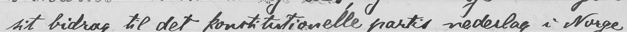sit bidrag til det konstitutionelle partis nederlag i Norge
